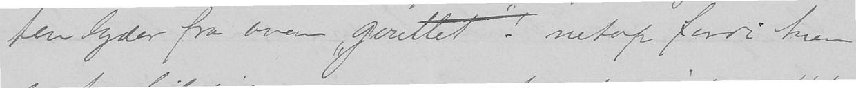ten lyder fra oven "gerettet"! netop fordi hun
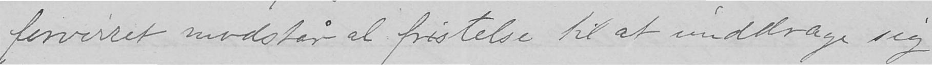forvirret modstår al fristelse til at unddrage sig
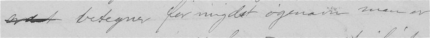ordet betegner for mig det øgenavn man er
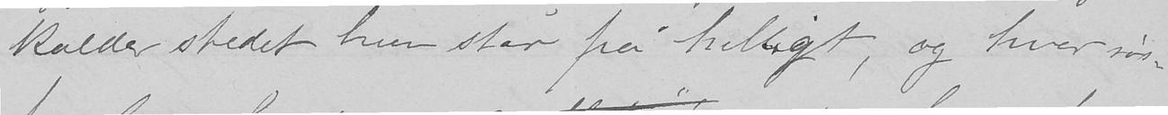kalder stedet hun står på helligt, og hvor røs-
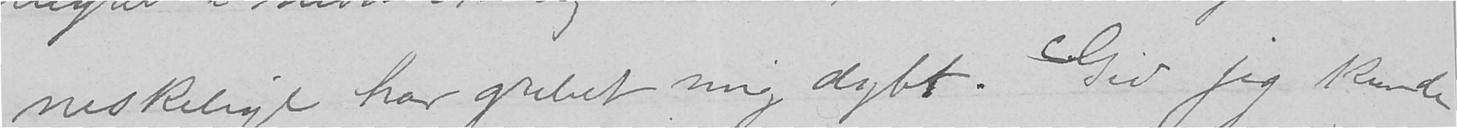neskelige har grebet mig dybt. Gid jeg kunde
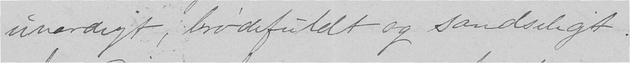uværdigt, brødefuldt og sandseligt.
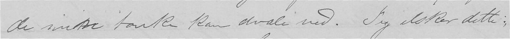de min tanke kan dvæle ved. Jeg elsker dette;
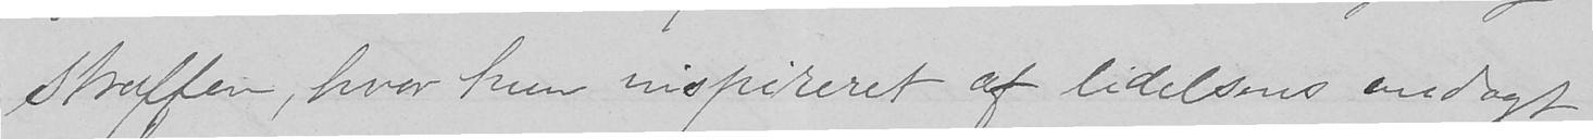straffen, hvor hun inspireret af lidelsens andagt
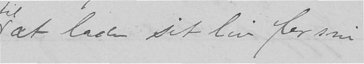at lade sit liv for sin
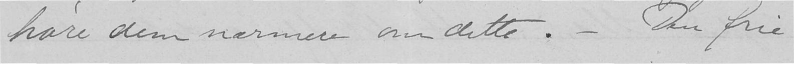høre dem nærmere om dette. - Den frie
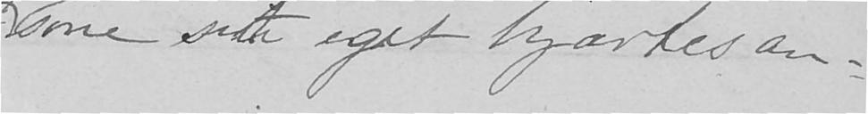sone sitt eget hjærtes an-
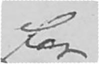for
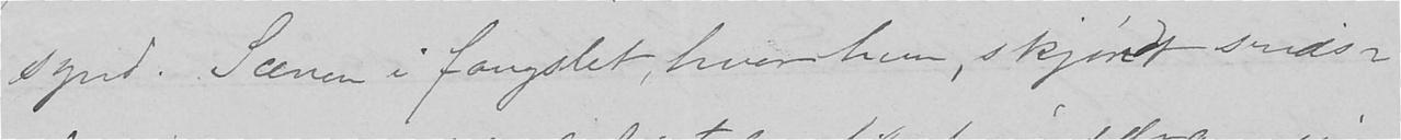synd. Scenen i fængslet, hvor hun, skjøndt sinds-
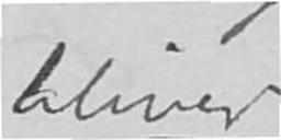bliver
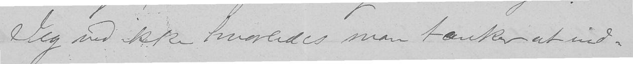Jeg ved ikke hvorledes man tænker at ind-
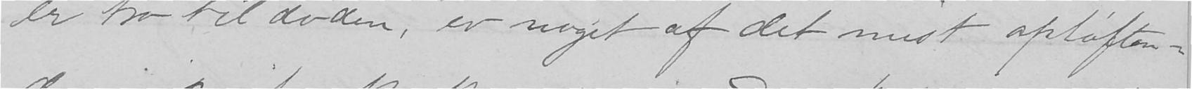er tro til døden, er noget af det mest opløften-
